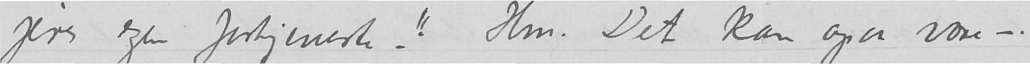jeres egen fortjeneste–».  Hm. Det kan ogsaa være -.
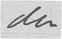din
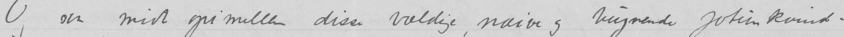Og saa midt opimellem disse vældige, naive og bugnende jotunkvindfolkene
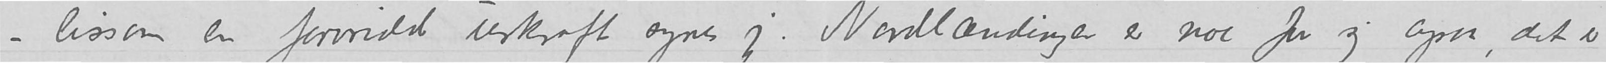lissom en forvridd urkraft synes jeg. Nordlændinger er noe for sig ogsaa, det er sandt!
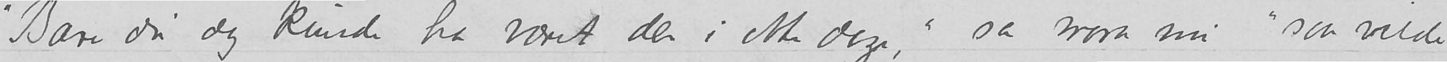«Bare du dog kunde ha været der i ett døgn,» sa mora mi.  «saa vilde du
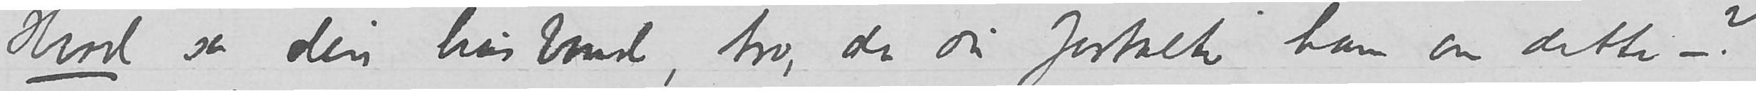Hvad sa din husbond, tro, da du fortalte ham om dette –?
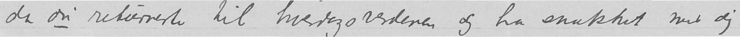da du returnerte til hverdagsverdenen og ha snakket med dig.
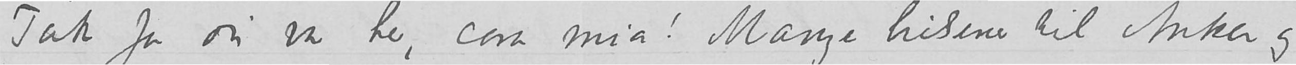Tak for du var her, cora mia!  Mange hilsener til Anker og
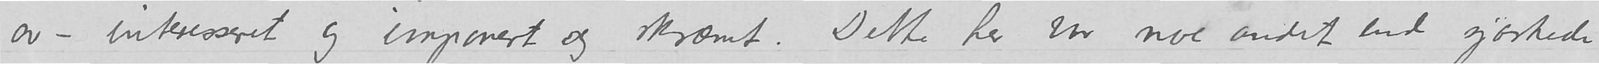av– interesseret og imponert og skræmt. Dette her var noe andet end sjuskede
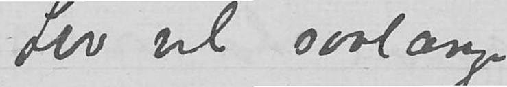Lev vel saalænge
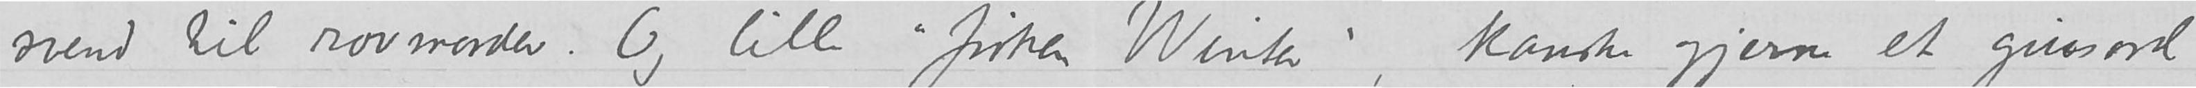svend til rovmorder.  Og lille «frøken Winter», kanske gjerne et gudsord
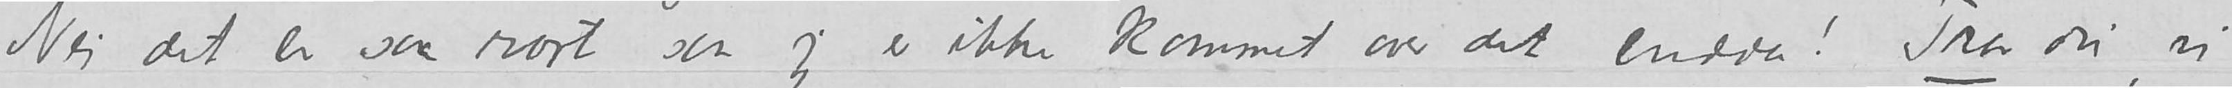Nei det er saa rart saa jeg er ikke kommet over det endda!  Tror du, vi
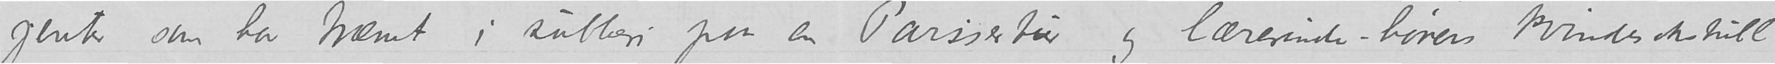jenter som har trænet i subberi paa en Parisertur og lærerinde- hørers kvindesakstull
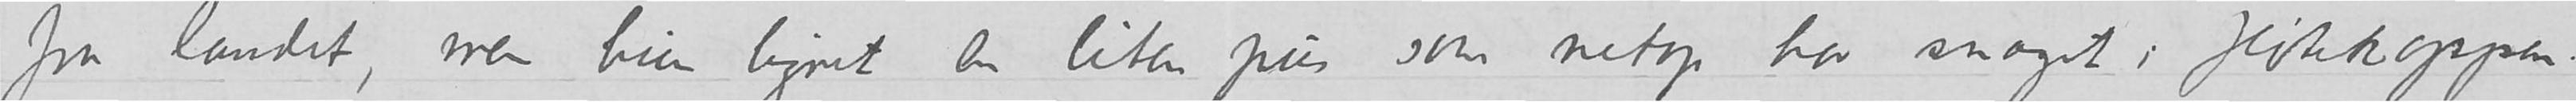fra landet, men hun lignet en liten pus som netop har snoget i fløtekoppen.
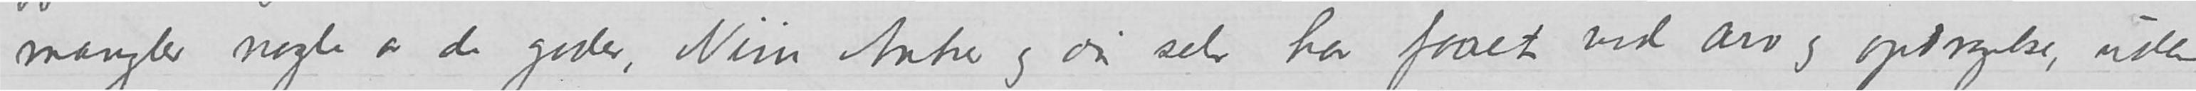mangler nogle av de goder, Nini Anker og du selv har faaet ved arv og opdragelse, uden
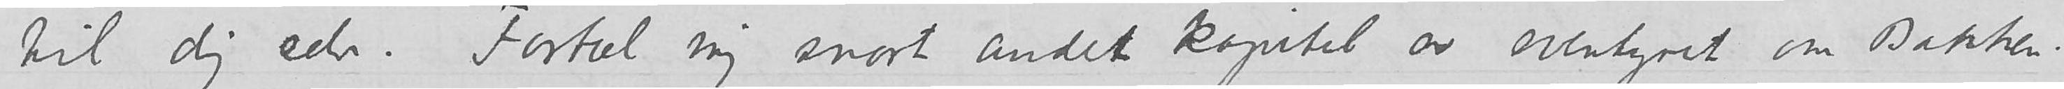til dig selv. Fortæl mig snart andet kapitel av eventyret om Bakken.
